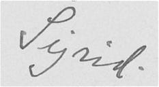Sigrid.
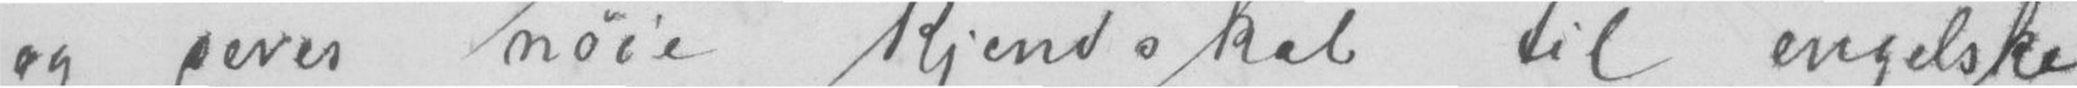og deres nøie Kjendskab til engelske
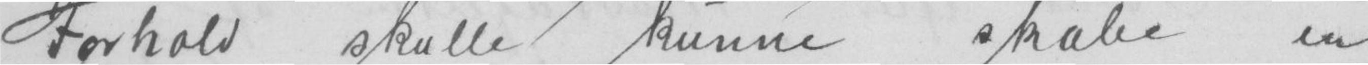Forhold skulle kunne skabe en
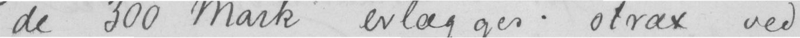de 300 Mark erlægges strax ved
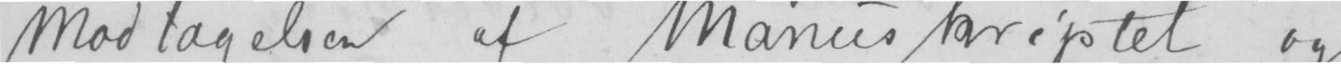Modtagelser af Manuskriptet og
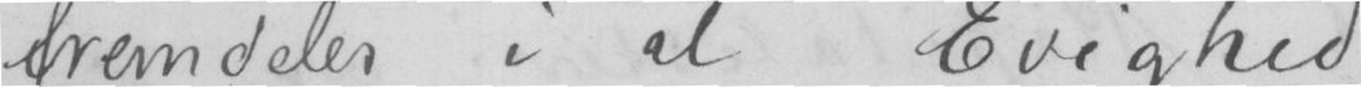fremdeles i al Evighed
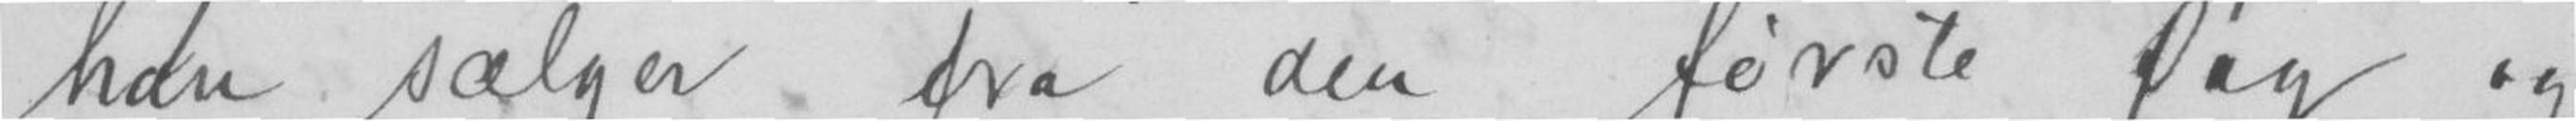han sælger fra den første Dag og
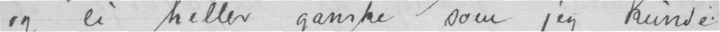og ei heller ganske som jeg kunde
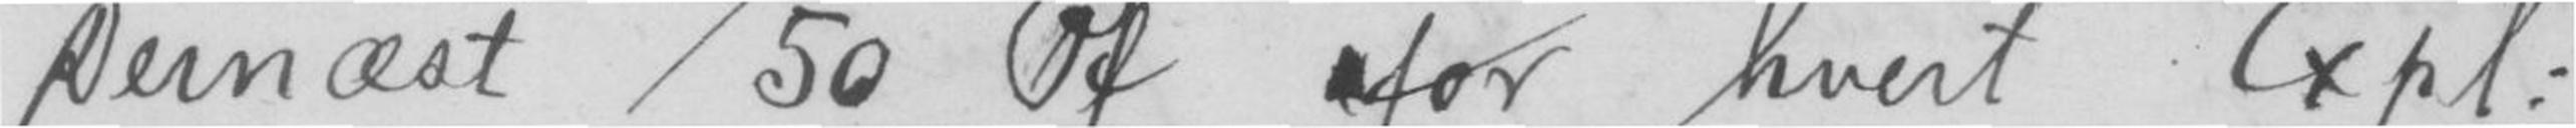Dernæst 50 Pt for hvert Expl:
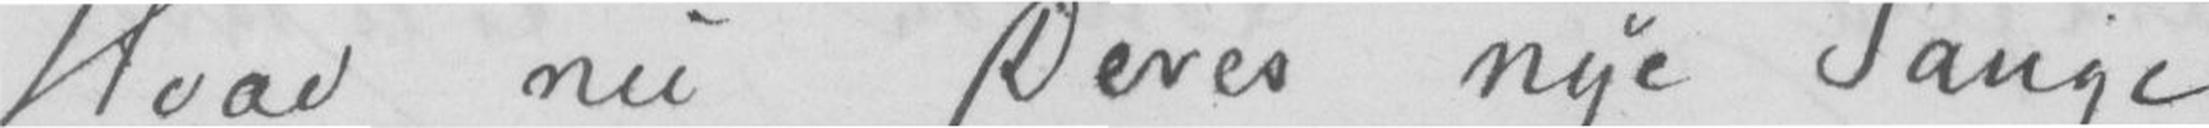Hvad nu Deres nye Sange
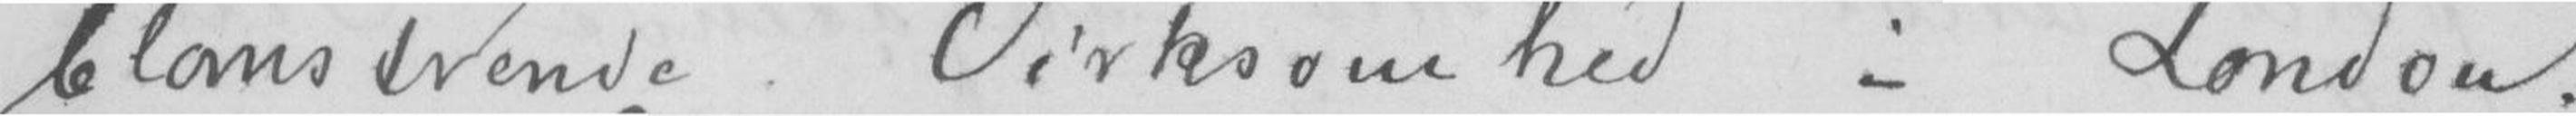blomstrende Virksomhed i London.
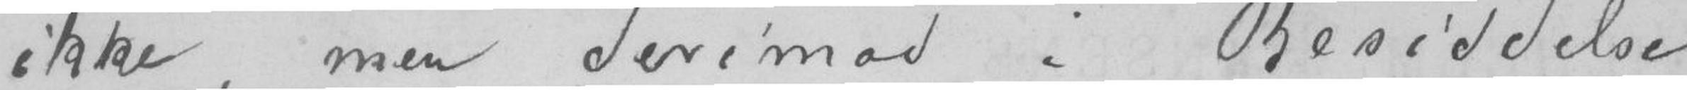ikke, men derimod i Besiddelse
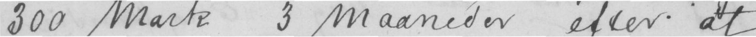300 Mark 3 Maaneder efter at
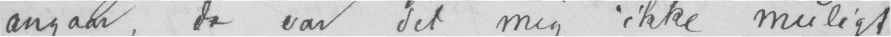angaar, da var det mig ikke muligt
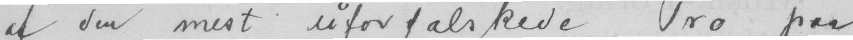af den mest uforfalskede Tro paa
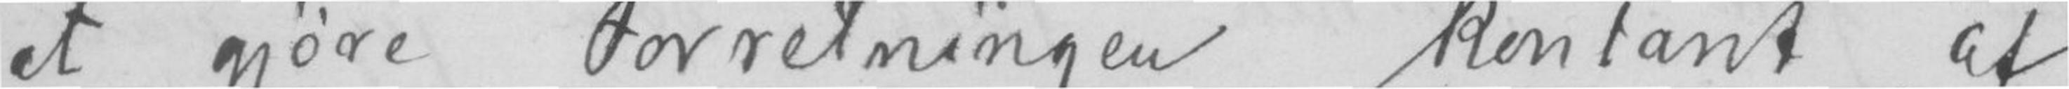at gjøre Forretningen Kontant af,
